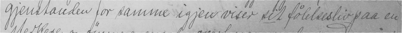gjenstanden for samme igjen viser sit følelsesliv paa en
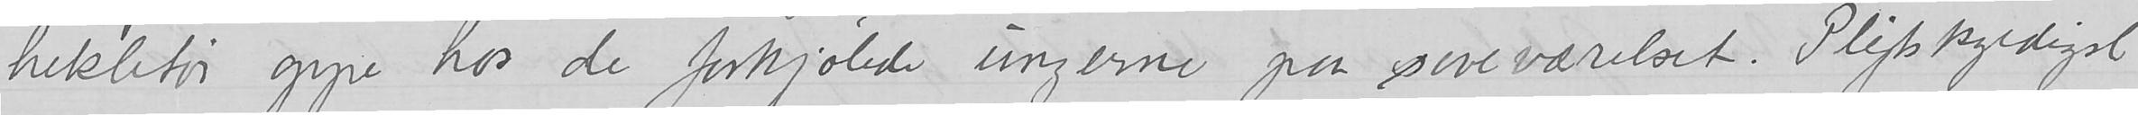hekletøi oppe hos de forkjølede ungerne paa soveværelset. Pligtskyldigst
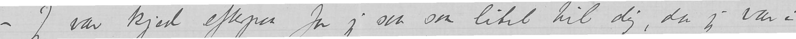– Jeg var kjed efterpaa for jeg saa saa litet til dig, da jeg var i
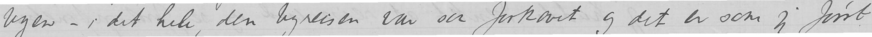byen – i det hele, den byreisen var saa forkavet og det er som jeg først
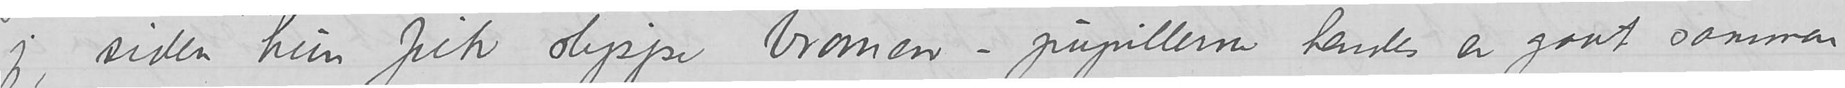jeg, siden hun fik slippe bromen – pupillerne hendes er gaat sammen
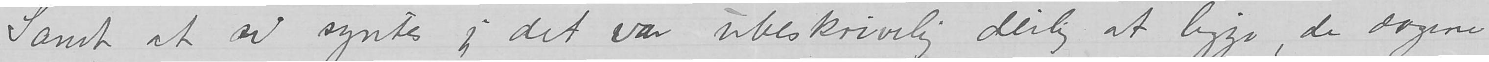Sandt at si syntes jeg det var ubeskrivelig deilig at ligge, de dagene
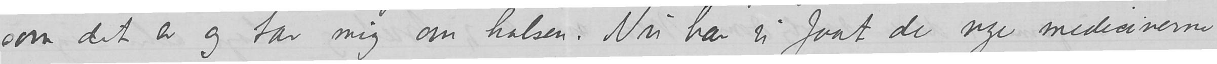som det er og tar mig om halsen. Nu har vi faat de nye medicinerne
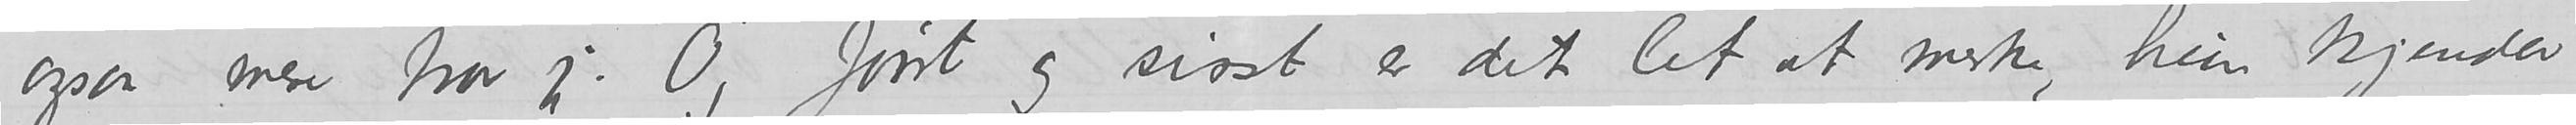ogsaa mere tror jeg. Og først og sisst er det let at merke, hun kjender
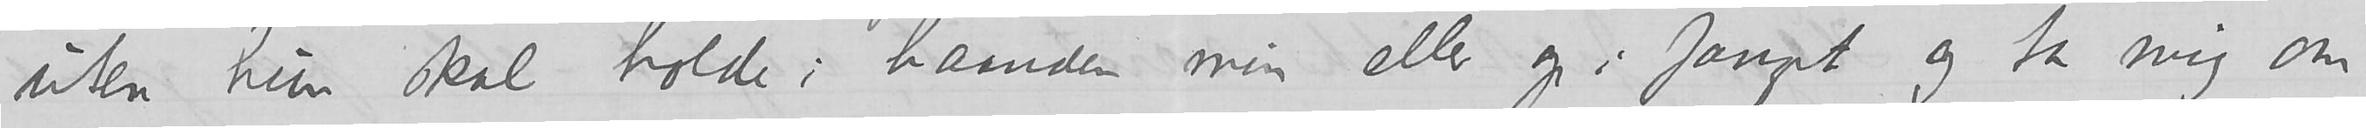uten hun skal holde i haanden min eller op i fanget og ta mig om
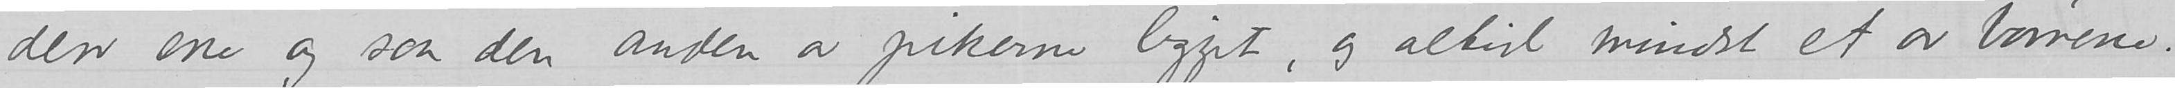den ene og saa den anden av pikerne ligget, og altid mindst et av børnene.
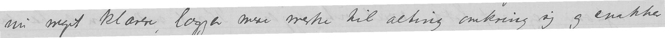nu meget klarere, lægger mere merke til alting omkring sig og snakker
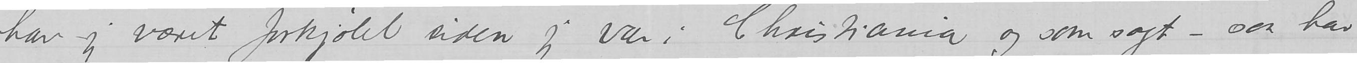har jeg været forkjølet siden jeg var i Christiania og som sagt – saa har
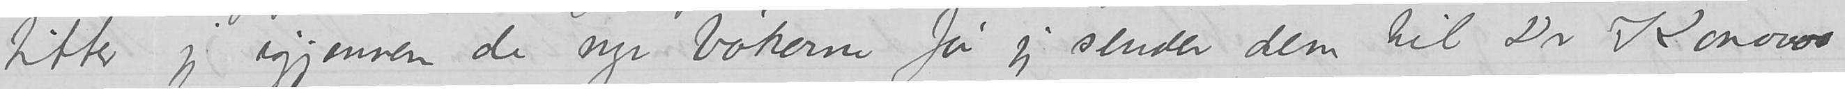titter jeg igjennem de nye bøkerne før jeg sender dem til Dr Konows
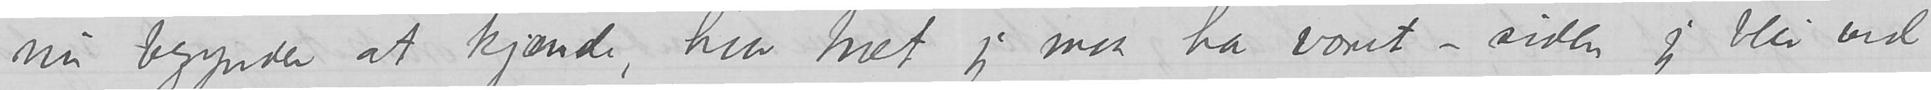nu begynder at kjænde, hvor træt jeg maa ha været – siden jeg blir ved
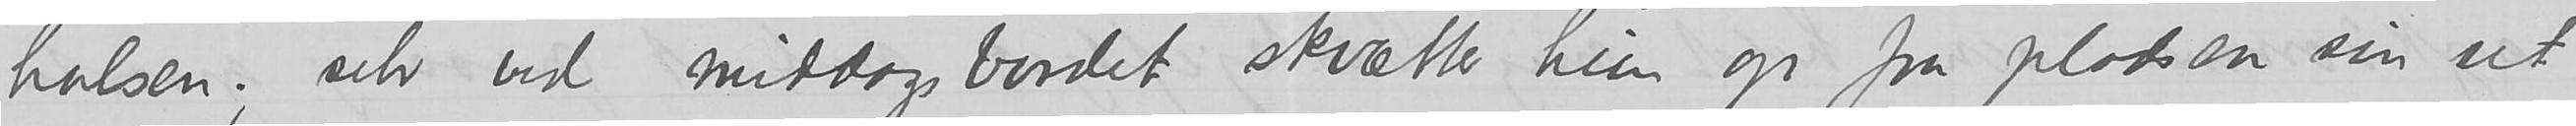halsen; selv ved middagsbordet skvætter hun op fra pladsen sin ret
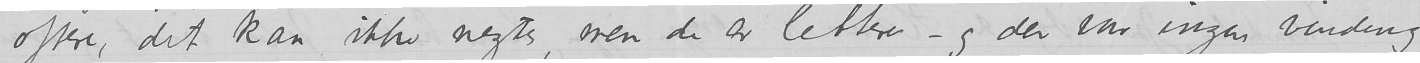oftere, det kan ikke negtes, men de er lettere – og der var ingen vinding
